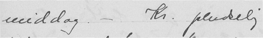middag. - Kr. pludselig
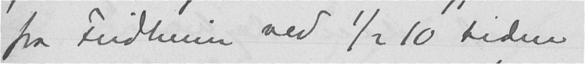fra Fridheim ved ½ 10 tiden
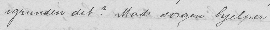igrunden det? Mod sorgen hjelper
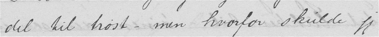del til trøst - men hvorfor skulde jeg
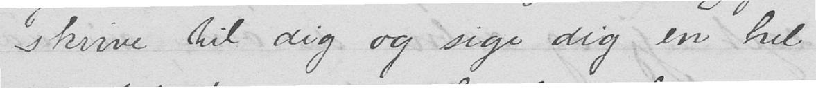skrive til dig og sige dig en hel
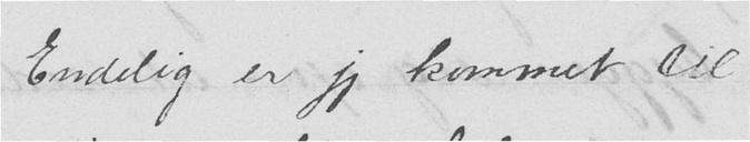Endelig er jeg kommet til
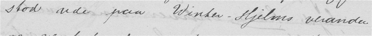stod ude paa Winter-Hjelms veranda
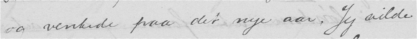og ventede paa det nye aar. Jeg vilde
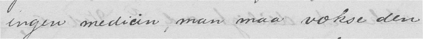ingen medicin, man maa vokse den
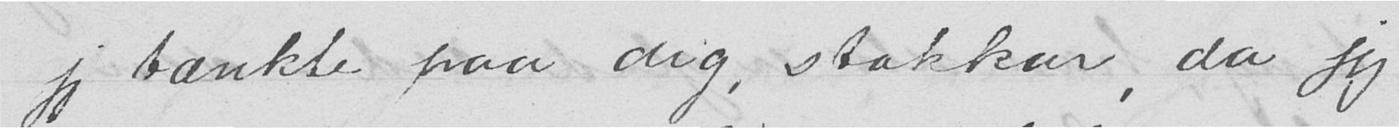jeg tænkte paa dig, stakkar, da jeg
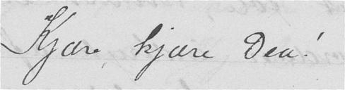Kjære, kjære Dea!
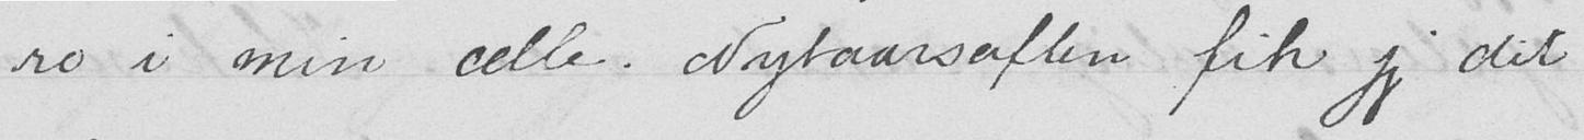ro i min celle. Nytaarsaften fik jeg dit
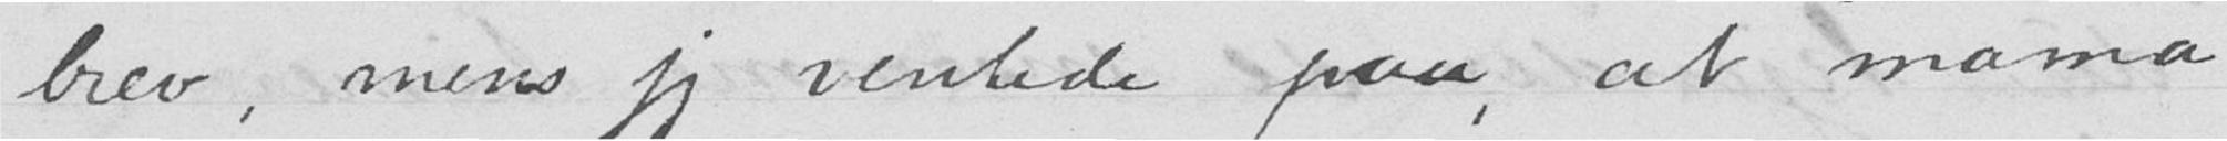brev, mens jeg ventede paa, at mama
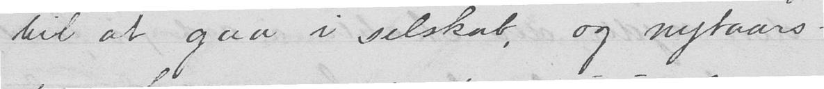til at gaa i selskab, og nytaars-
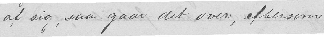at sig, saa gaar det over, eftersom
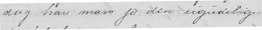dag har man jo den ugudelige
